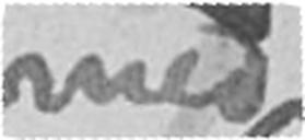med
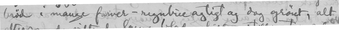brød i mange farver - regnbueagtigt og dog grønt, alt
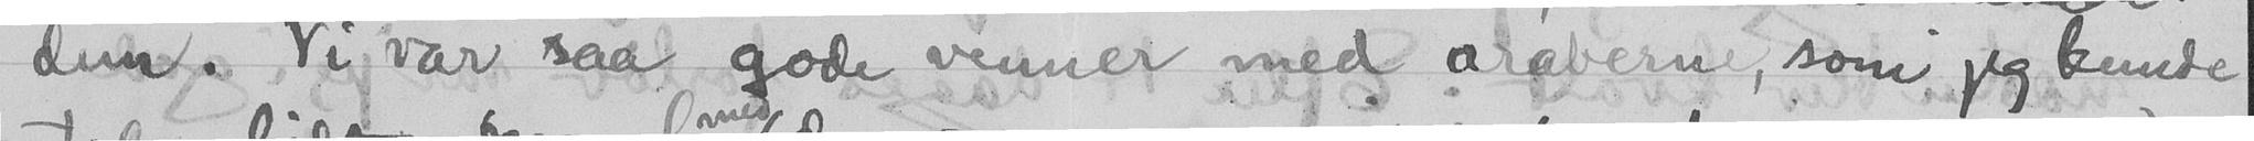den. Vi var saa gode venner med araberne, som jeg kunde
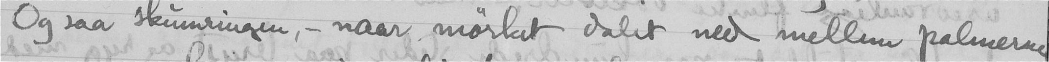Og saa skumringen, - naar mørket dalet ned mellem palmerne
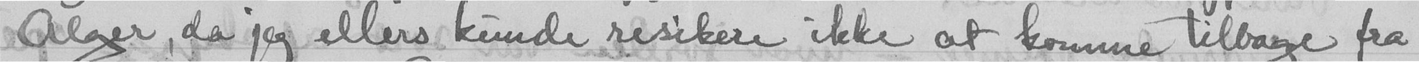Alger, da jeg ellers kunde resikere ikke at komme tilbage fra
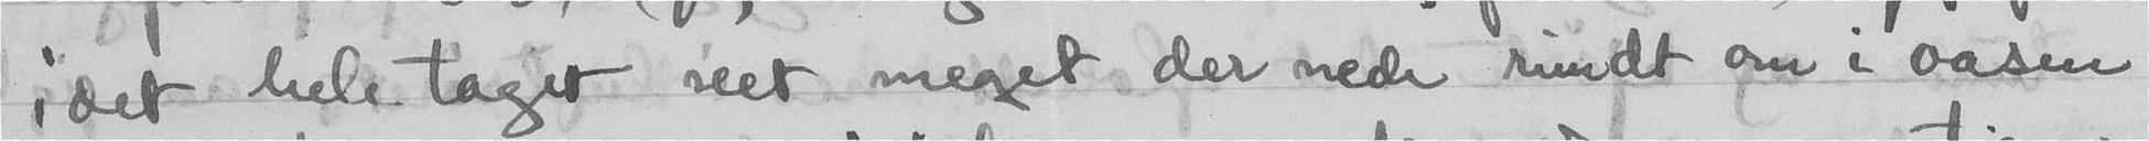i det hele taget seet meget der nede rundt om i oasen
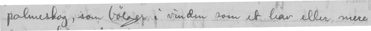palmeskog, som bølger i vinden som et hav eller mere
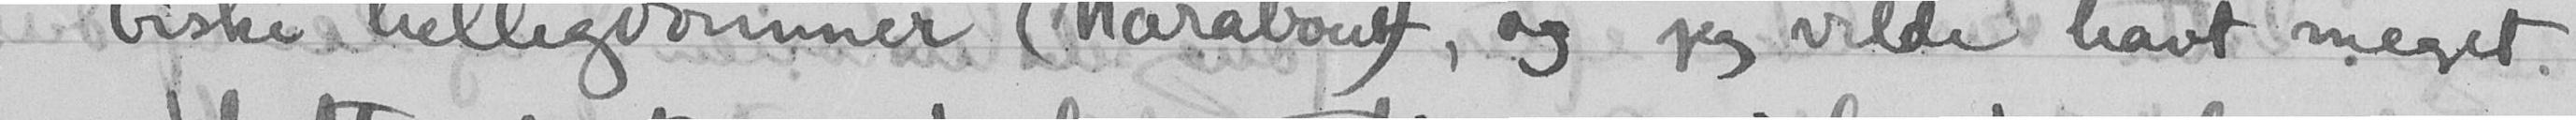biske helligdommer (Marabont), og jeg vilde havt meget
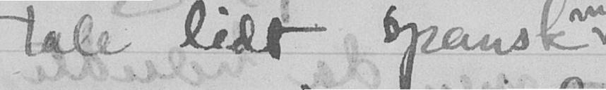tale lidt spansk
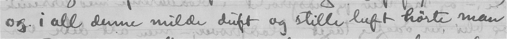og i all denne milde duft og stille luft hørte man
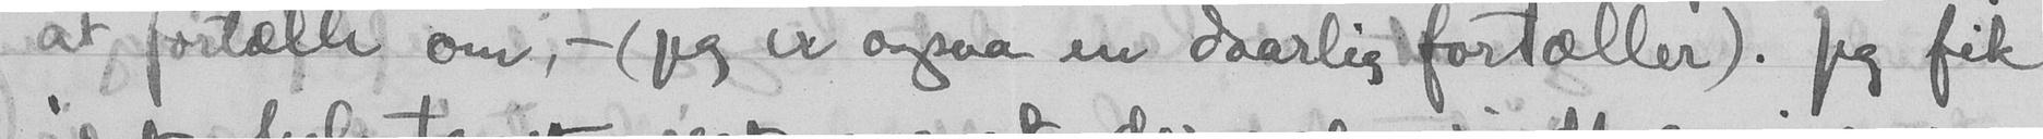at fortælle om, - (jeg er ogsaa en daarlig fortæller). Jeg fik
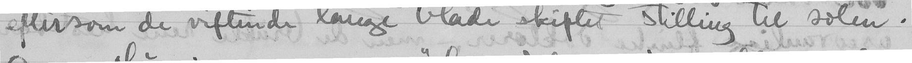eftersom de viftende lange blade skiftet stilling til solen.
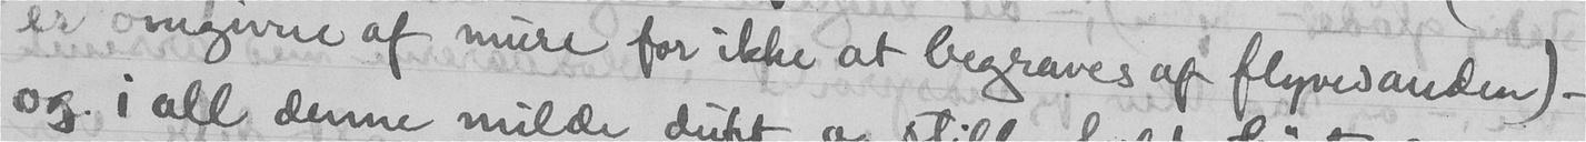er omgivne af mure for ikke at begraves af flyvesanden) -
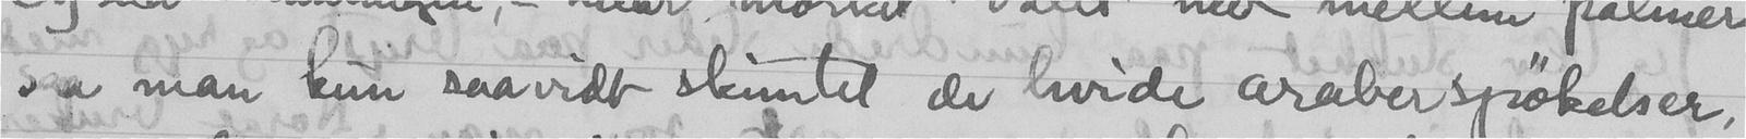saa man kun saavidt skimtet de hvide araberspøkelser,
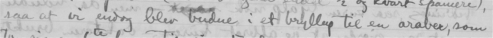saa at vi endog blev budne i et bryllup til en araber, som
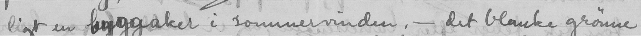ligt en byggaker i sommervinden, - det blanke grønne
